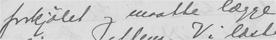forkjølet og maatte lægge
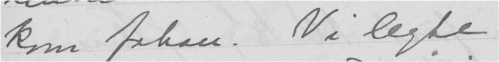kom Johan. Vi legte
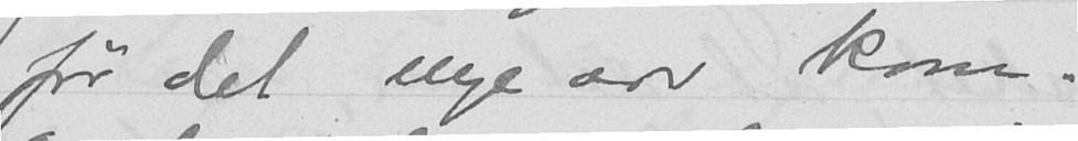før det nye aar kom.
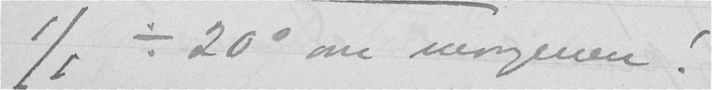1/1 ÷ 20º om morgenen!
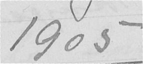1905
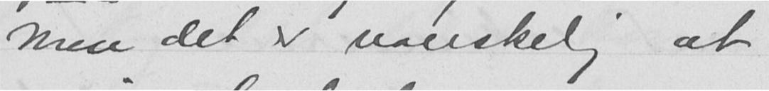men det er vanskelig at
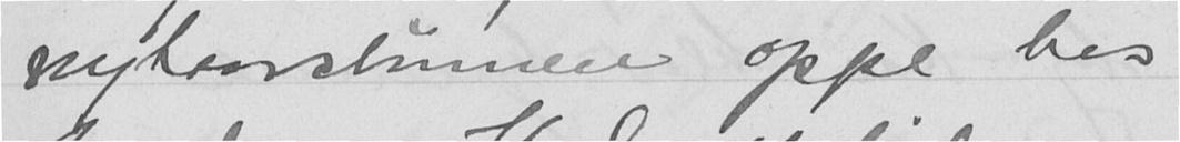nytaarsbønnen oppe hos
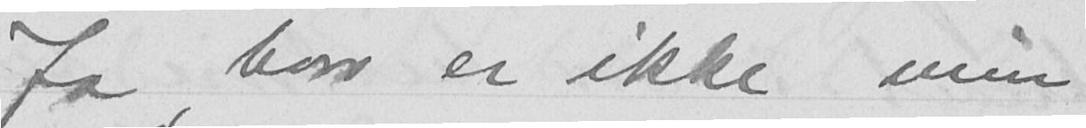Ja, hvor er ikke min
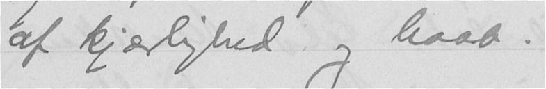af kjærlighed og haab.
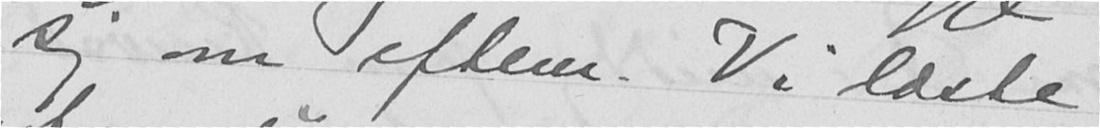sig om efterm. Vi læste
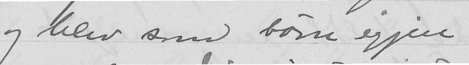og blev som børn igjen,
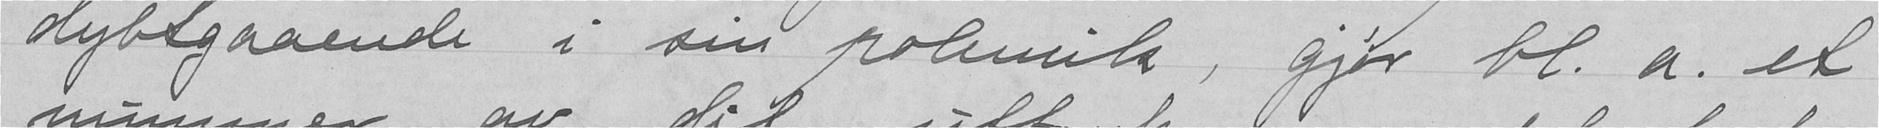dybtgaaende i sin polemik, gjør bl.a. et
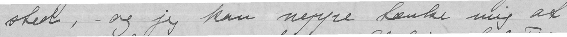sted, og jeg kan neppe tænke mig at
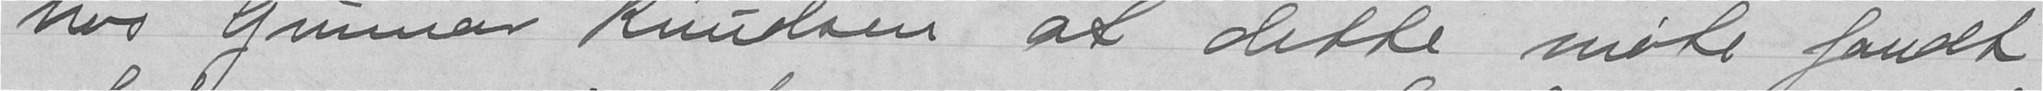hos Gunnar Knudsen at dette møte fandt
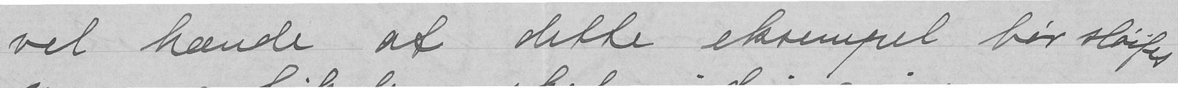vil hænde at dette eksempel bør sløifes
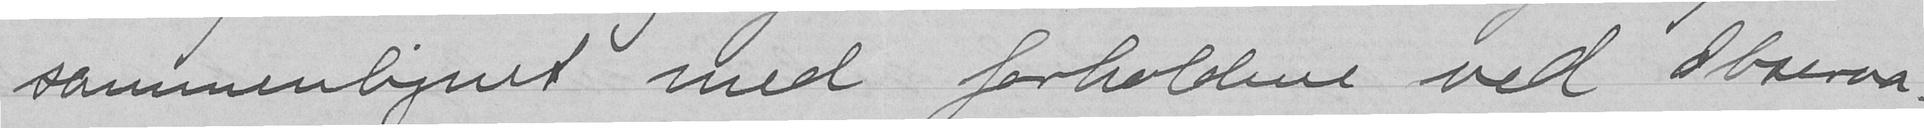sammenlignet med forholdene ved Observa-
